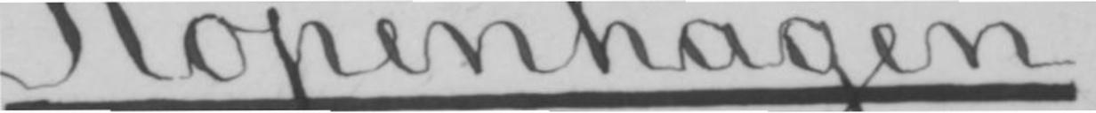Kopenhagen.
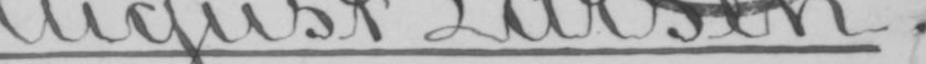August Larsen.
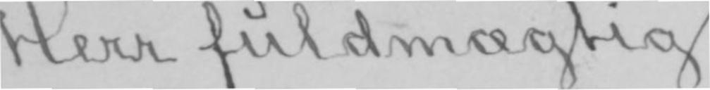Herr fuldmægtig
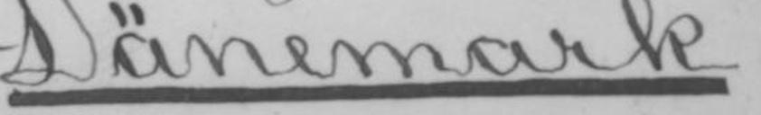Dänemark.
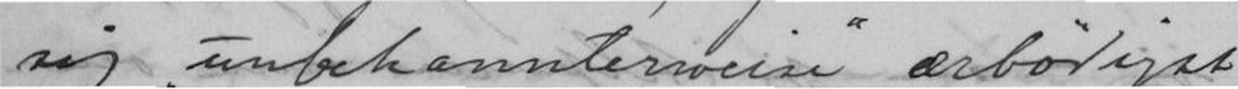sig unbekannterweise ærbødigst
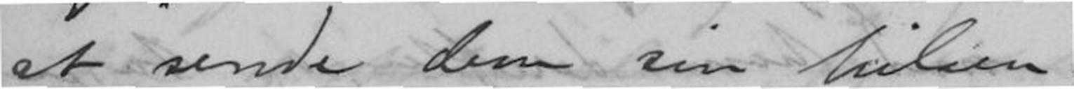at sende dem sin hilsen.
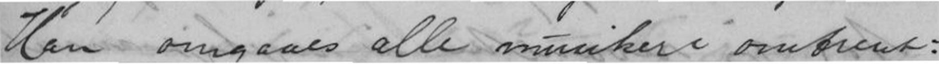Han omgaaes alle musikere omtrent:
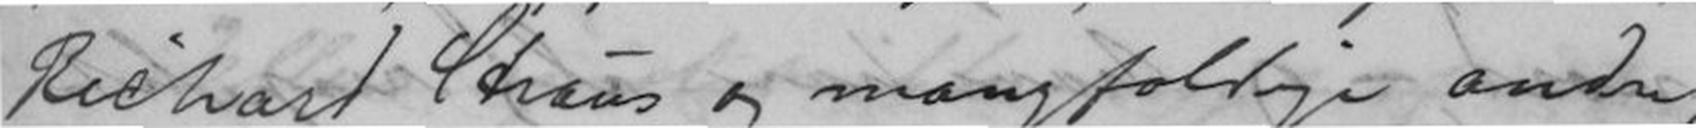Richard Straus og mangfoldige andre,
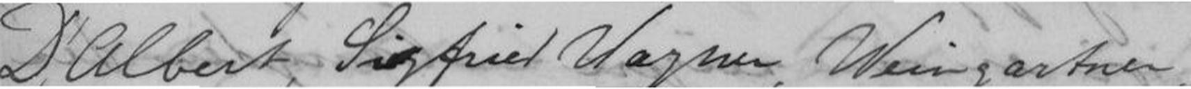D'Albert, Sigfried Wagner, Weingartner,
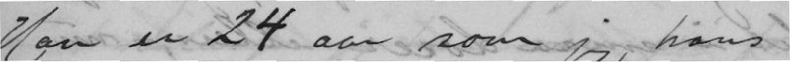Han er 24 aar som jeg, hans
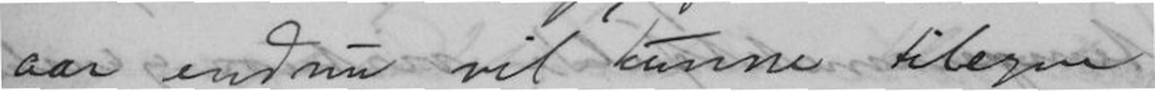aar endnu vil kunne tilegne
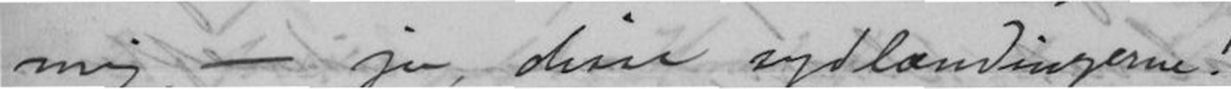mig, - ja, disse sydlændingerne!
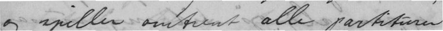og spiller omtrent alle partiturer
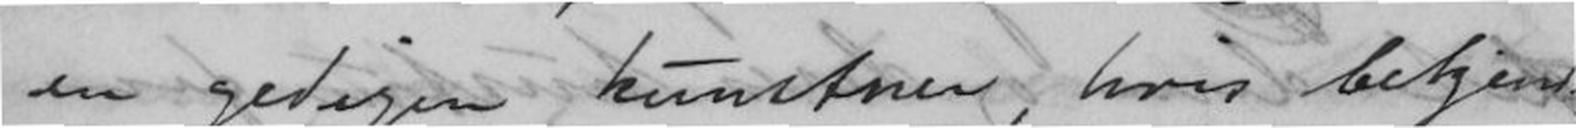en gedigen kunstner, hvis bekjendt-
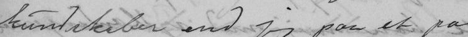kundskaber end jeg paa et par
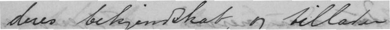deres bekjendskab, og tillader
